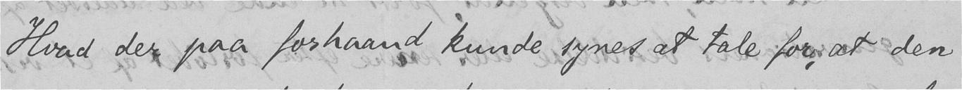Hvad der paa forhaand kunde synes at tale for, at den
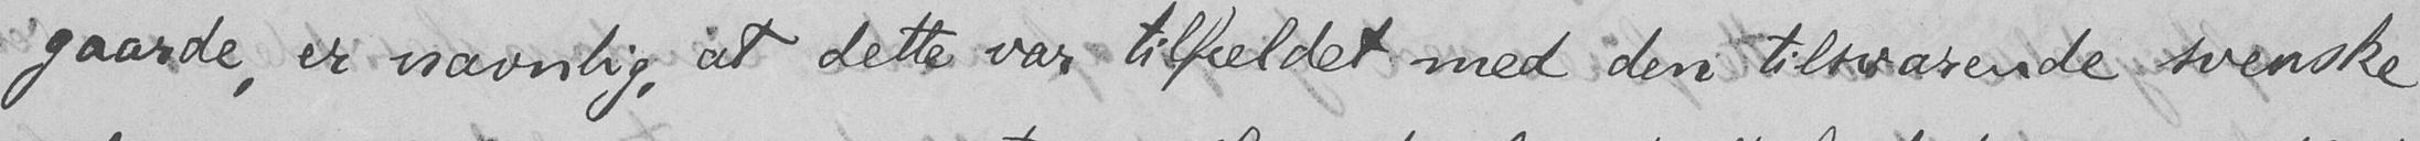gaarde, er navnlig, at dette var tilfældet med den tilsvarende svenske
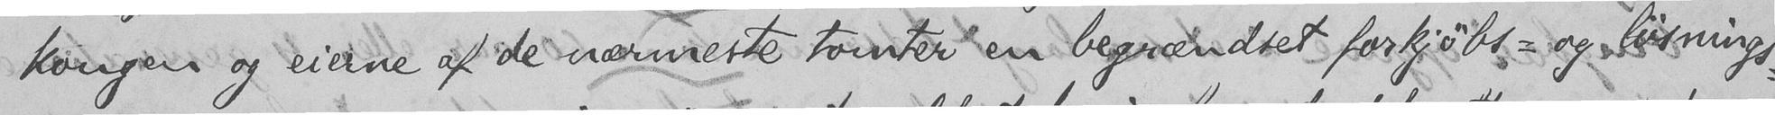kongen og eierne af de nærmeste tomter en begrændset forkjøbs- og løsnings-
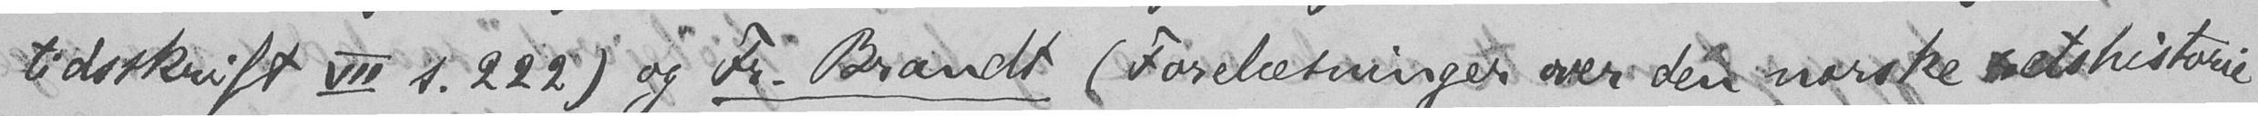tidsskrift VII s. 222) og Fr. Brandt (Forelæsninger over den norske retshistorie
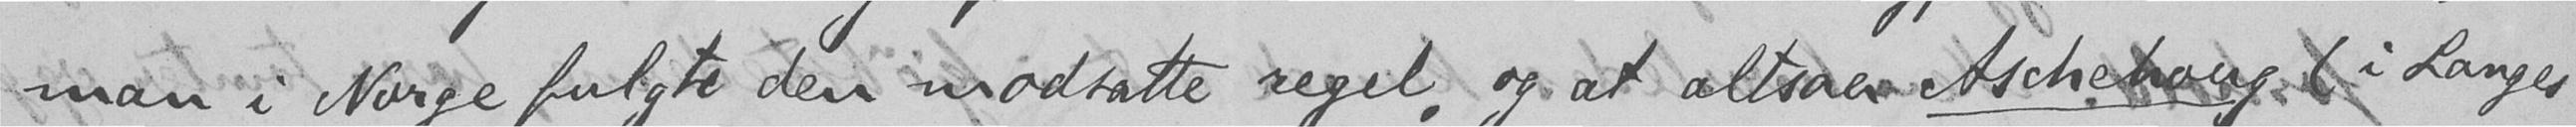man i Norge fulgte den modsatte regel, og at altsaa Aschehoug (i Langes
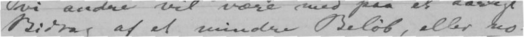Bidrag af et mindre Beløb, eller no-
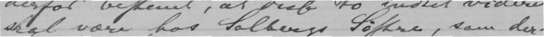skal være hos Solbergs Søstre, som der-
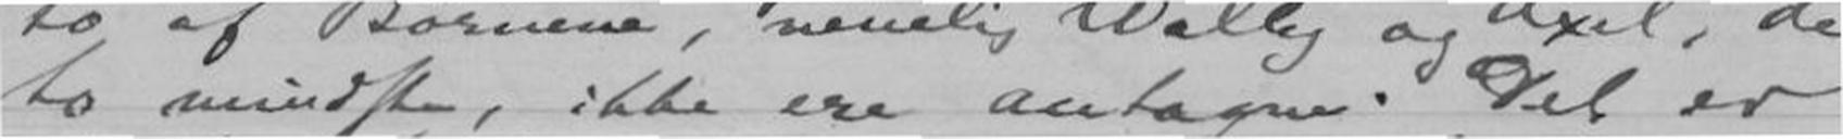to mindste, ikke ere antagne. Det er
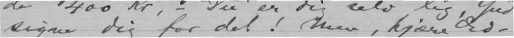signe dig for det! Men,kjære Ed-
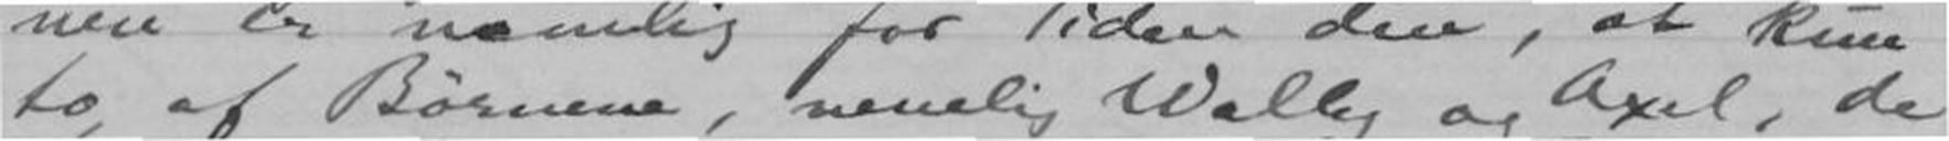to af Børnene, nemlig Wally og Axel, de
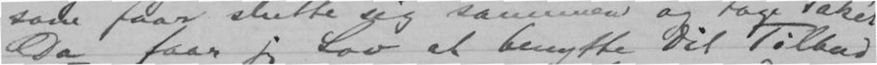Da faar jeg Lov at benytte Dit Tilbud
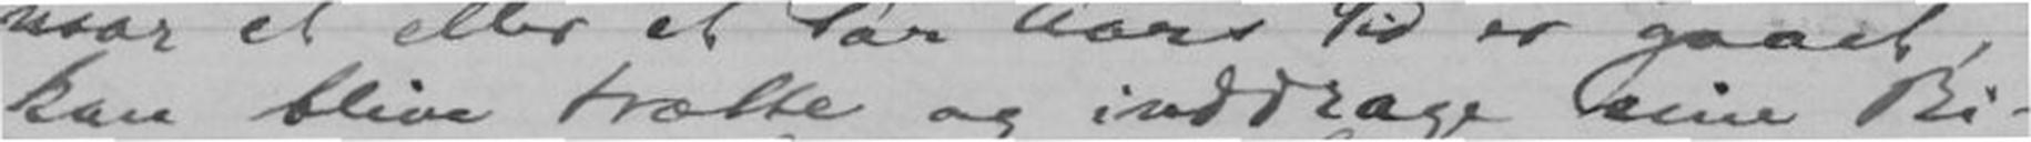kan blive trætte og inddrage sine Bi-
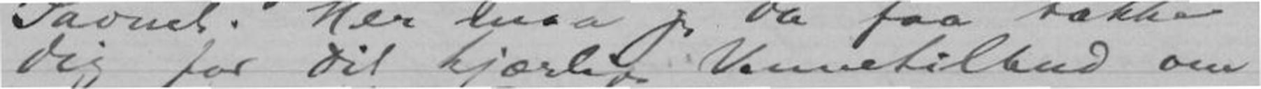Dig for Dit kjærlige Vennetilbud om
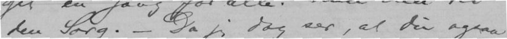den Sorg. - Da jeg dog ser, at Du ogsaa
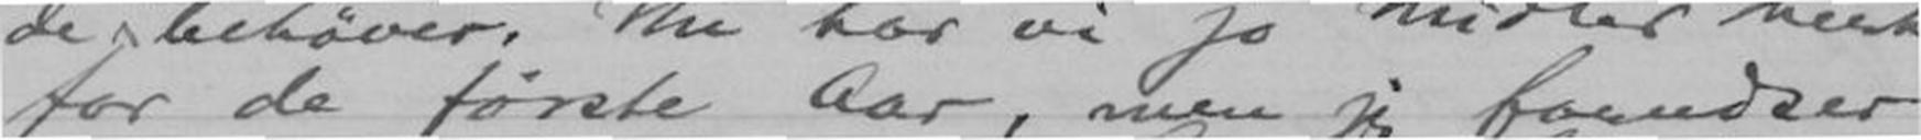for de første Aar, men jeg forudser
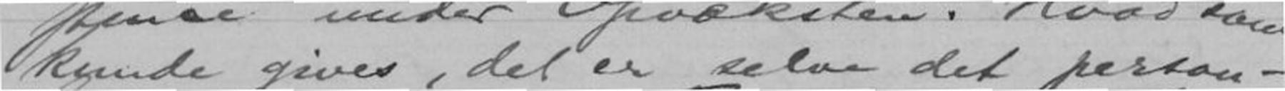kunde gives, det er selve det person-
